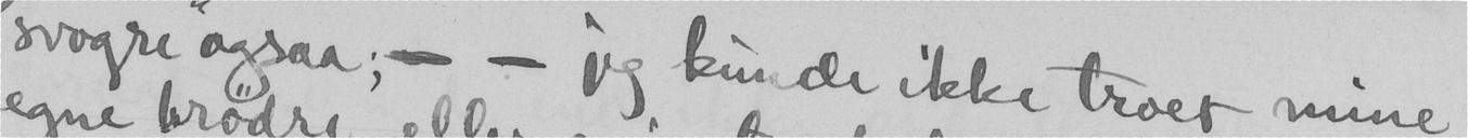"svogre" ogsaa. - - jeg kunde ikke troet mine
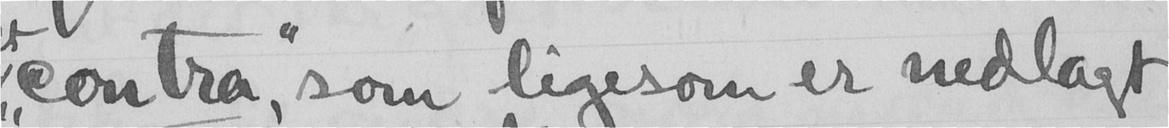"contra", som ligesom er nedlagt
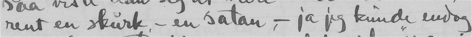rent en skurk - en satan, - ja jeg kunde endog
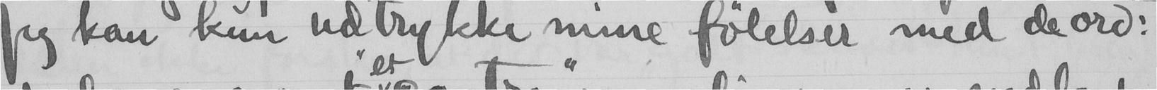Jeg kan kun udtrykke mine følelser med de ord:
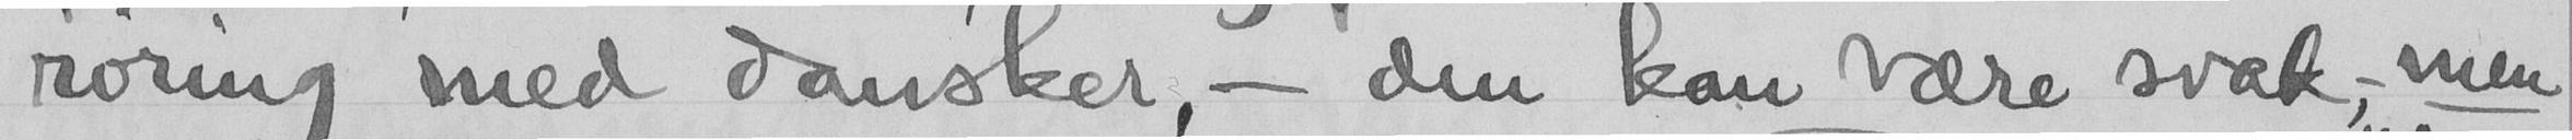rørig med dansker, - den kan være svak, men
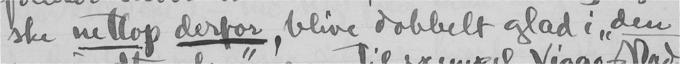ske nettop derfor blive dobbelt glad i "den
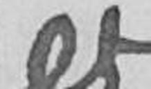et
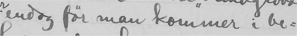endog før man kommer i be-
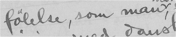følelse, som man,
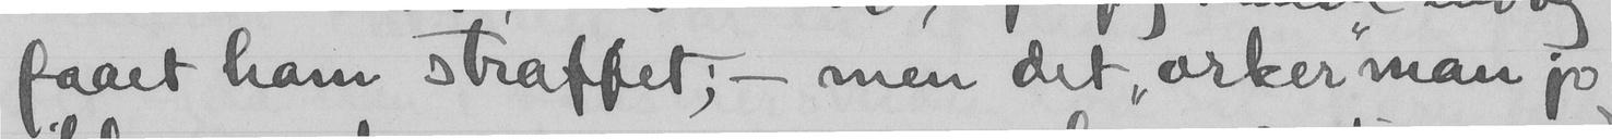faaet ham straffet, - men det "orker" man jo
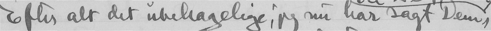Efter alt det ubehagelige, jeg nu har sagt Dem,
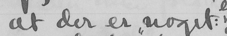at der er "noget":
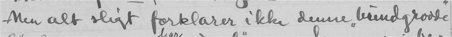Men alt sligt forklarer ikke denne "bundgrodde"
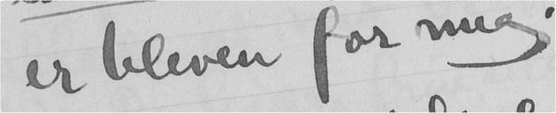er bleven for mig.
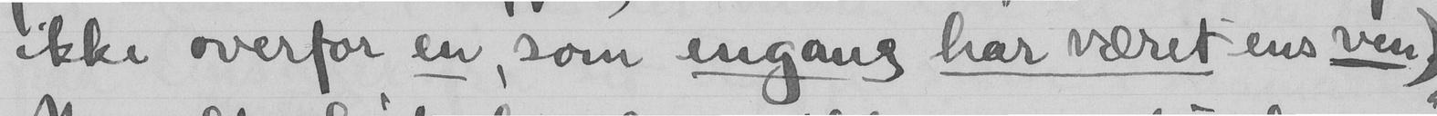ikke overfor en, som engang har været ens ven)
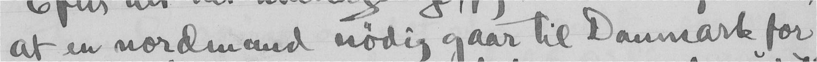at en nordmand nødig gaar til Danmark for
